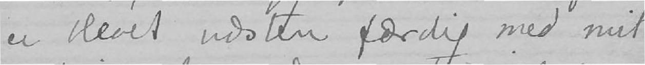er blevet næsten færdig med mit
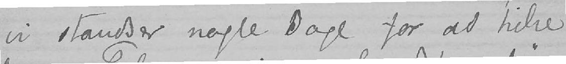vi standser nogle Dage for at hilse
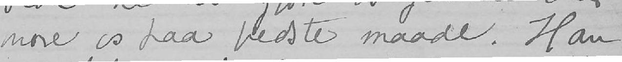more os paa bedste maade. Han
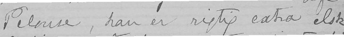Pelouse, han er rigtig extra elsk-
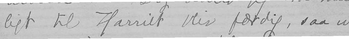ligt til Harriet blir færdig, saa vi
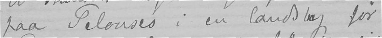paa Pelouses i en landsby før
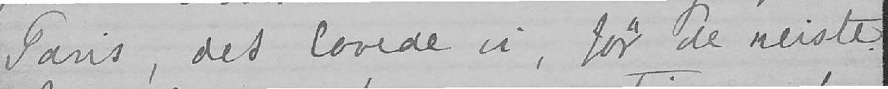Paris, det lovede vi, før de reiste.
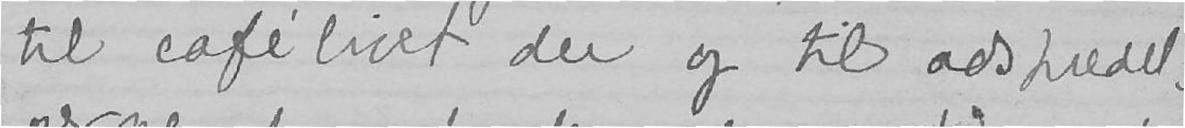til cafélivet der og til adspredel-
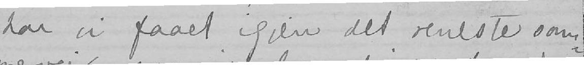har vi faaet igjen det reneste som-
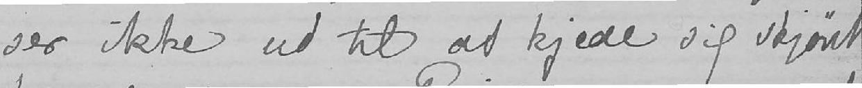ser ikke ud til at kjede sig skjønt
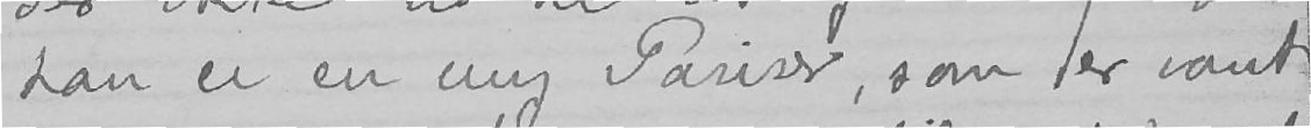han er en ung Pariser, som er vant
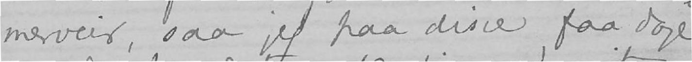merveir, saa jeg paa disse faa dage
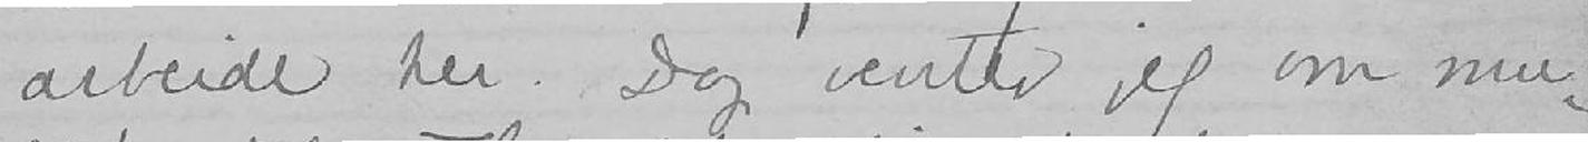arbeide her. Dog venter jeg om mu-
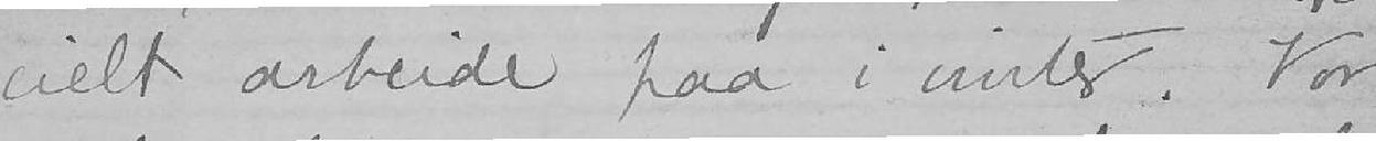cielt arbeide paa i vinter. Vor
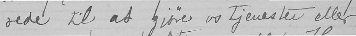rede til at gjøre os tjenester eller
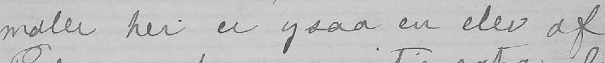maler her er ogsaa en elev af
